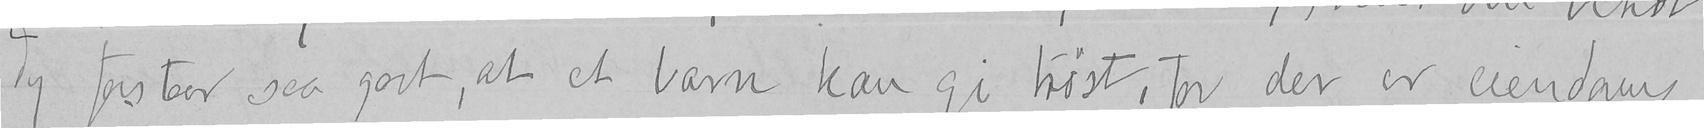Jeg forstaar saa godt, at et barn kan gi trøst, for der er eiendom,
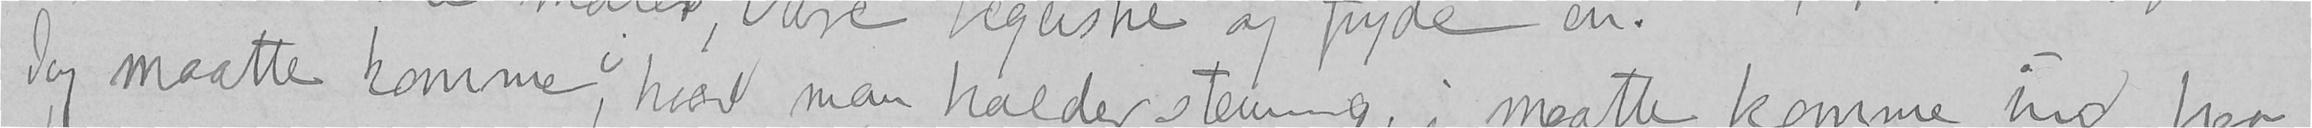Jeg maatte komme i, hvad man kalder stemning, jeg maatte komme ind paa
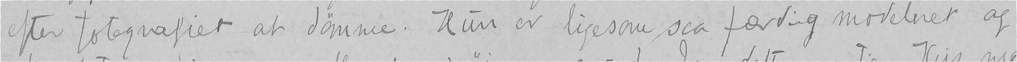efter fotografiet at dømme. Hun er ligesom saa færdig modeleret og
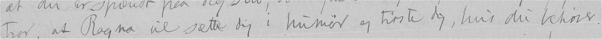tror, at Ragna vil sætte dig i humør og trøste dig, hvis du behøver.
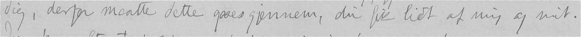dig, derfor maatte dette gaaes gjennem, du fik lidt af mig og mit.
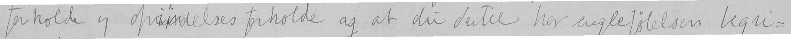forholde og oprindelses forholde, og at du dertil har englefølelsen begri-
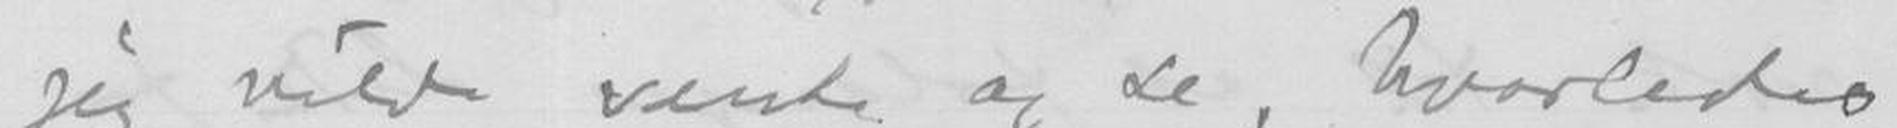jeg vilde vente og se, hvorledes
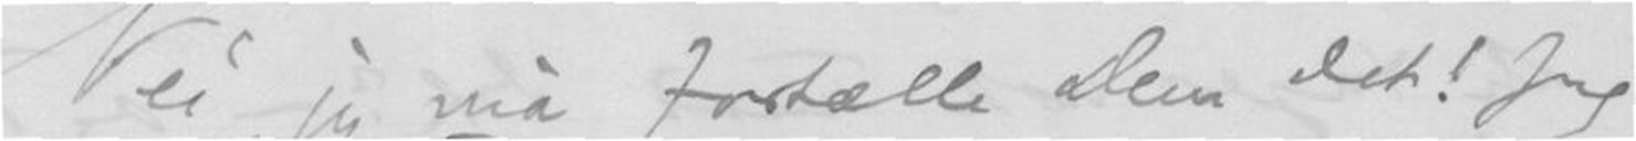Nei jeg må fortælle Dem det! Jeg
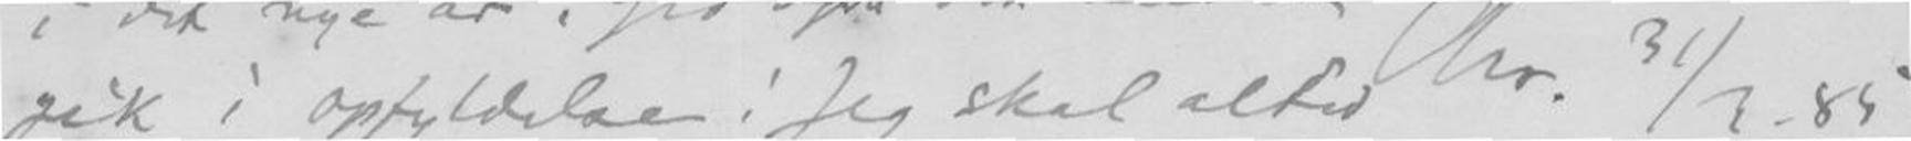gik i opfyldelse! Jeg skal altid Chr. 31/3.85
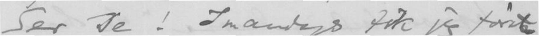Ser De! I mandags fik jeg første
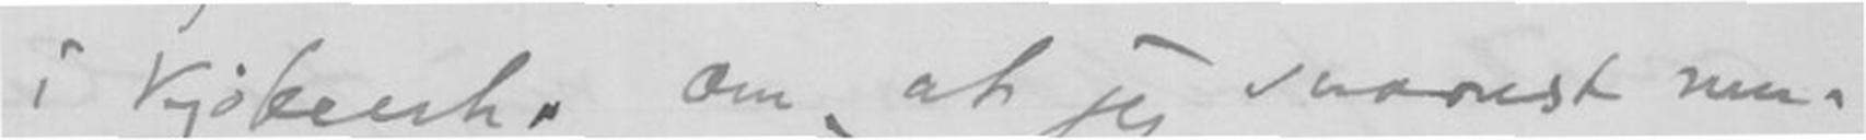i Kjøbenh. om, at jeg snarest mu-
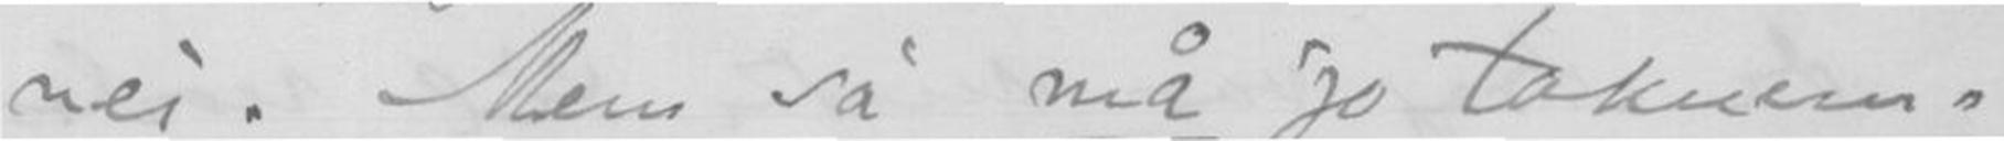nei. Men så må jo taknem-
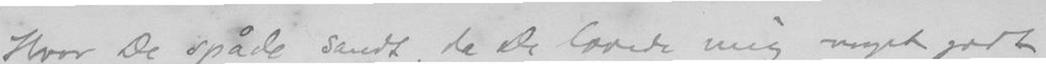Hvor De spåde sandt, da De lovede mig meget godt
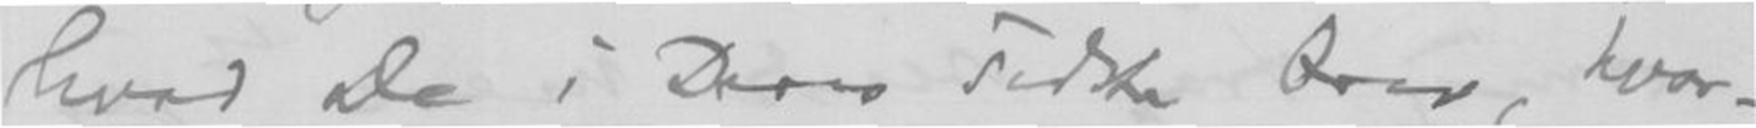hvad De i Deres sidste Brev, hvor-
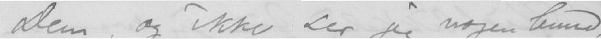Dem, og ikke ser jeg nogen bund,
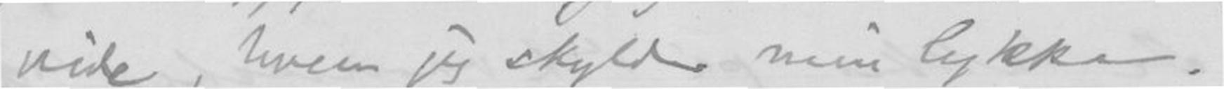vide, hvem jeg skylder min lykke.
